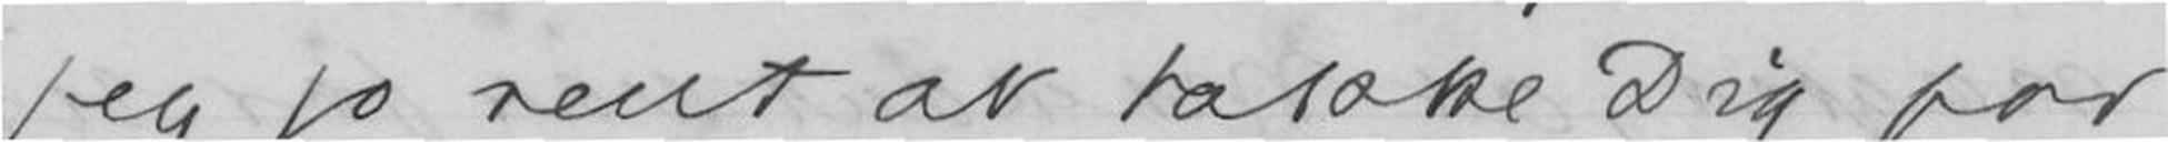jeg jo rent at takke Dig for
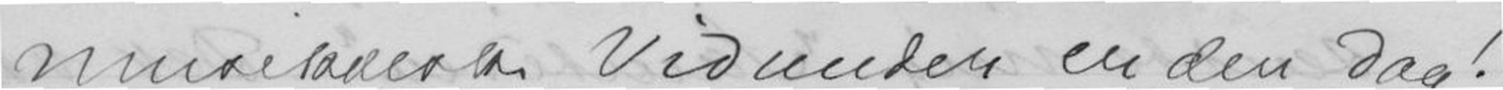musik. Vidunder er den dog!
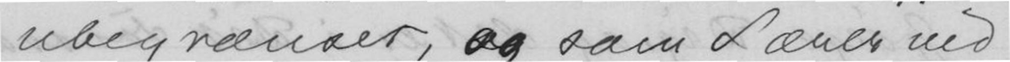ubegrænset, og som Lærer ved
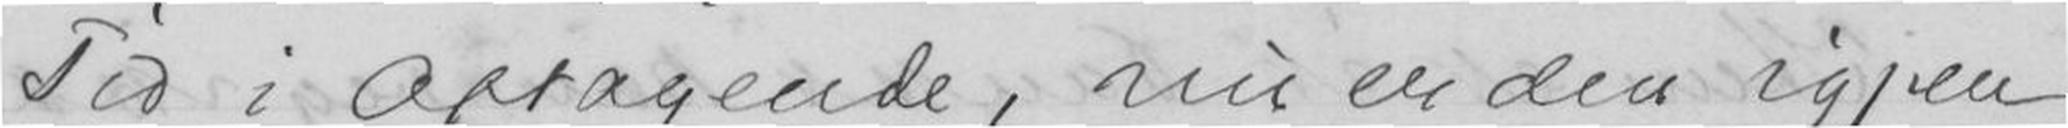Tid i Opragende, nu er den igjen
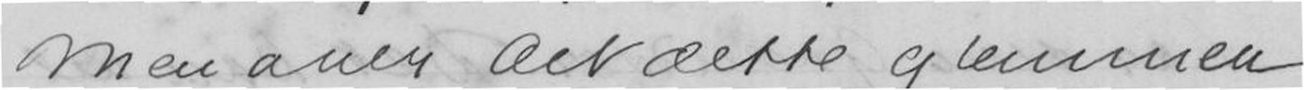Men over Alt dette glemmer
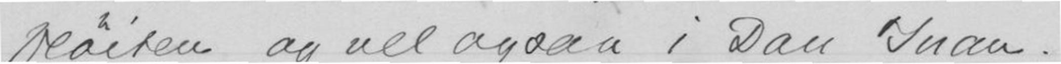fløiten og vel ogsaa i Don Juan.
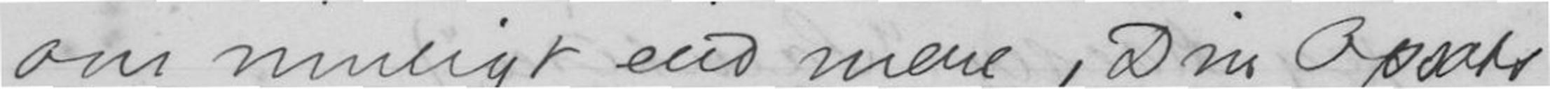om muligt end mere, Din Opsats
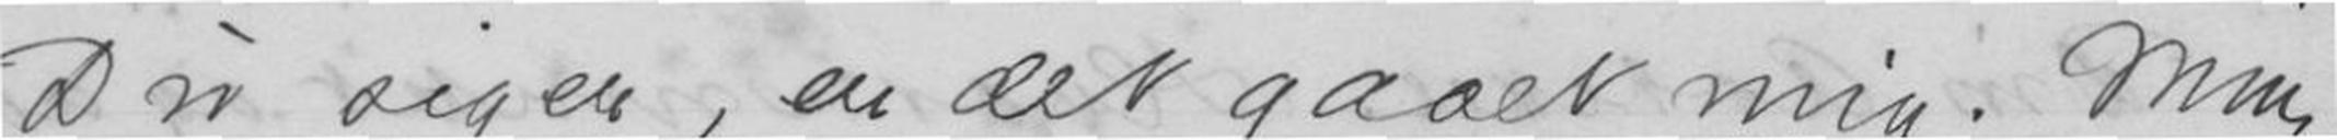Du siger, er det gaaet med mig. Min
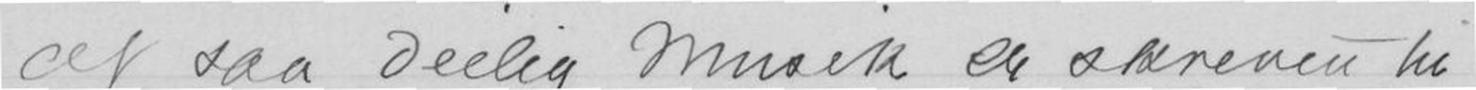At saa Deilig Musik er skreven til
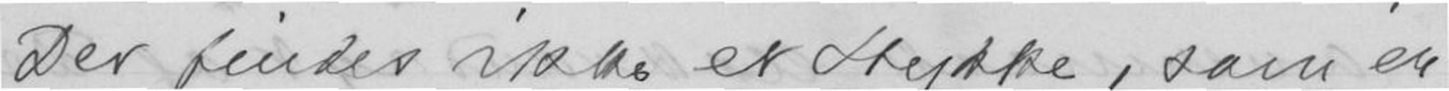Der findes ikke et stykke, som er
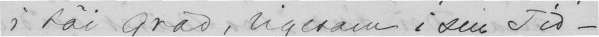i høi grad, ligesaa i sin Tid-
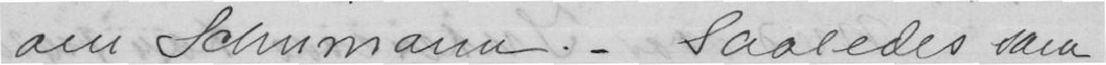om Schumann.- Saaledes som
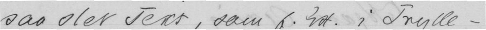saa slet Text, som f.eks. i Trylle-
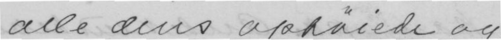alle dens ophøiede og
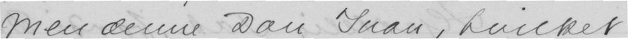Men denne Don Juan, hvilken
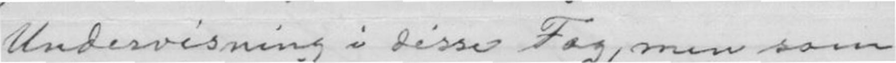Undervisning i disse Fag, men som
